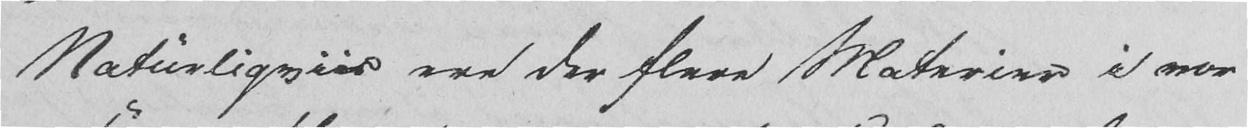Naturligviis ere der flere Materier i vor
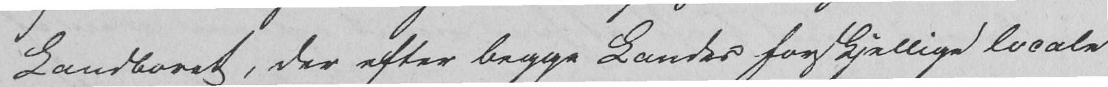Landboret, der efter begge Landes forskjellige locale
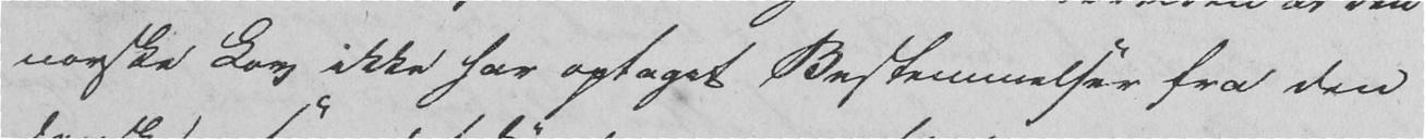norske Lov ikke har optaget Bestemmelser fra den
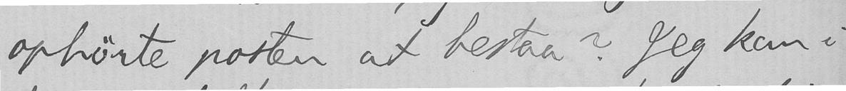ophørte posten at bestaa? Jeg kan i
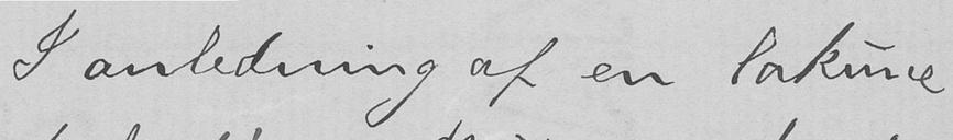I anledning af en lakune
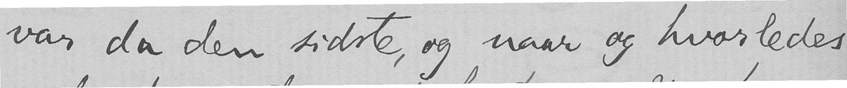var da den sidste, og naar og hvorledes
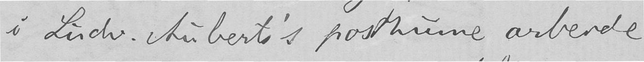i Ludv. Auberts's posthume arbeide
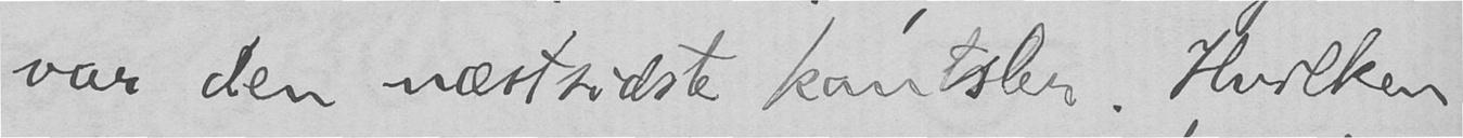var den næstsidste kantsler. Hvilken
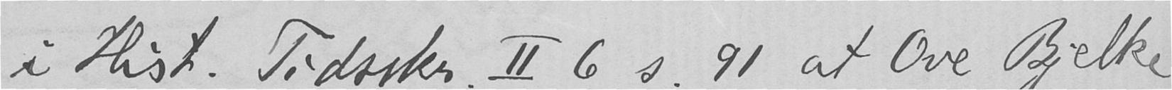i Hist. Tidsskr. II 6 s. 91, at Ove Bjelke
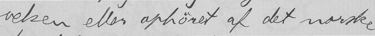velsen eller ophøret af det norske
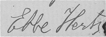Ebbe Hertz
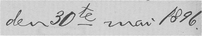den 30te mai 1896.
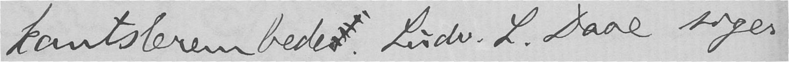kantslerembede. Ludv. L. Daae siger
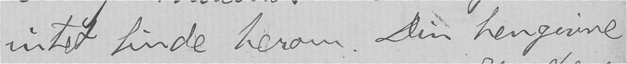intet finde herom. Din hengivne
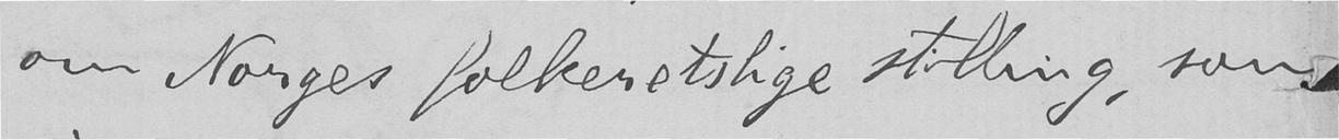om Norges folkeretslige stilling, som
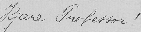Kjære Professor!
